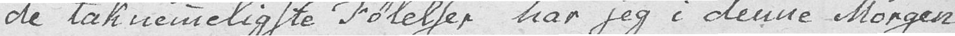de taknemmeligste Følelser har jeg i denne Morgen
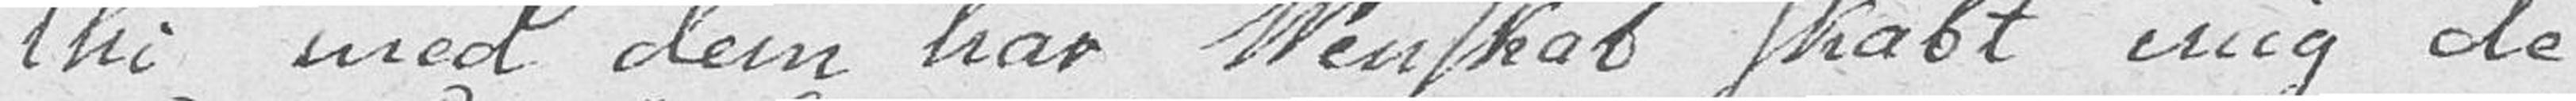thi med dem har Venskab skabt mig de
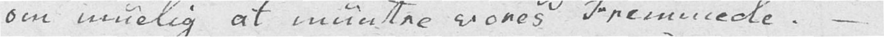om muelig at muntre vores Fremmede. –
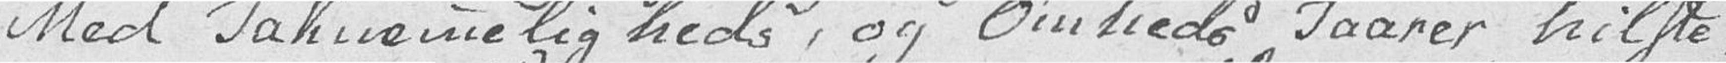Med Taknemmeligheds, og Ømheds Taarer hilste
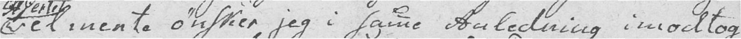Hjertets velmente ønsker jeg i samme Anledning imodtog
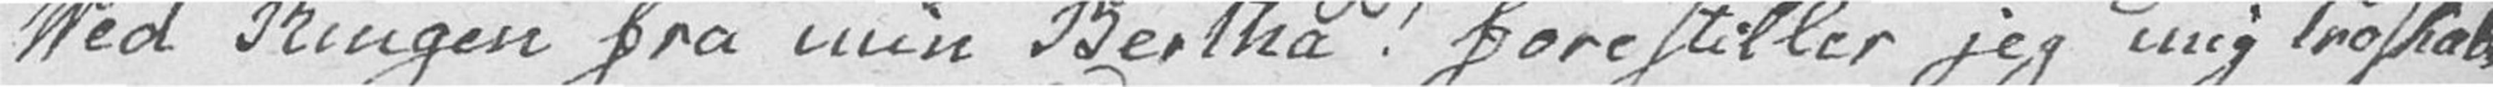Ved ringen fra min Bertha! forestiller jeg mig troskabs
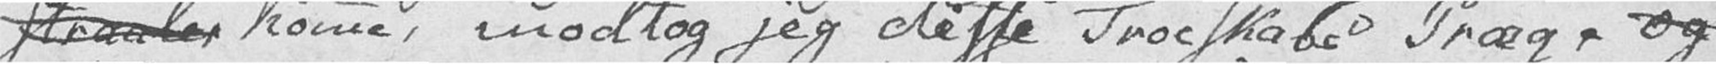straaler komme, modtog jeg dette Troeskabs Præg. og
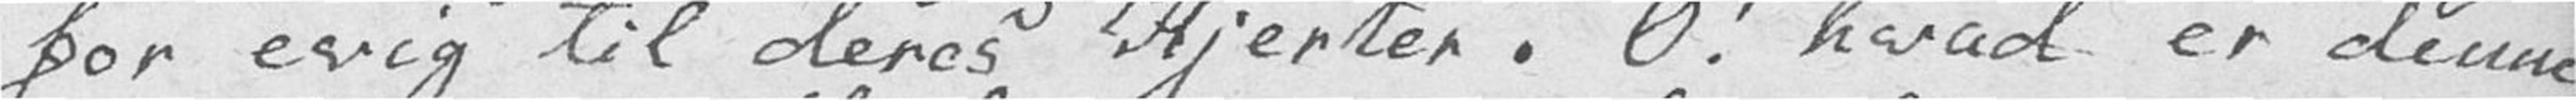for evig til deres Hjerter. O! hvad er denne
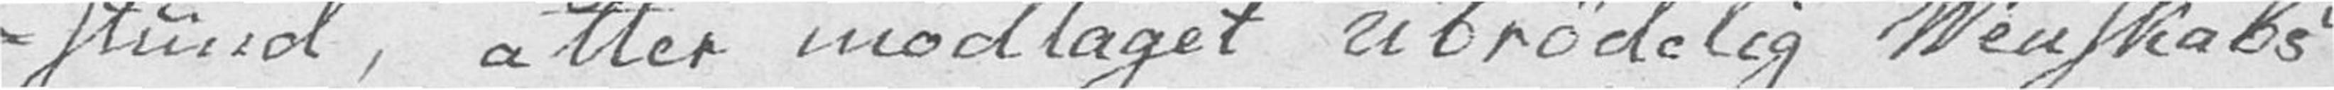-stund, atter modtaget ubrødelig Venskabs
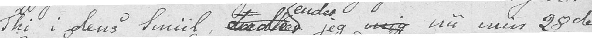Thi i dens Smiil  ender jeg mig nu min 28de
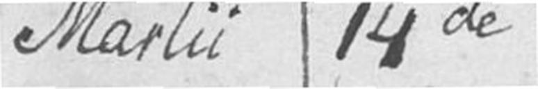Martii 14de
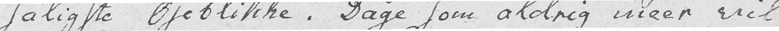saligste Øjeblikke! Dage som aldrig meer vil
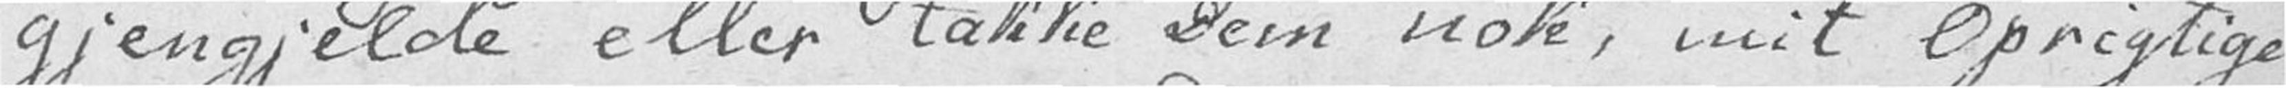gjengjelde eller takke Dem nok, mit Oprigtige
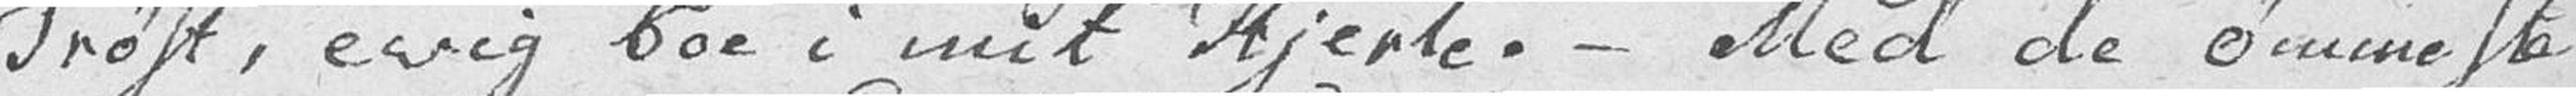Trøst, evig boe i mit Hjerte. – Med de ømmeste
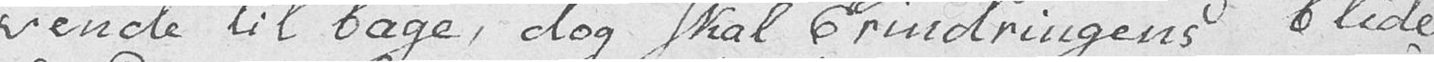vende til bage, dog skal Erindringens blide
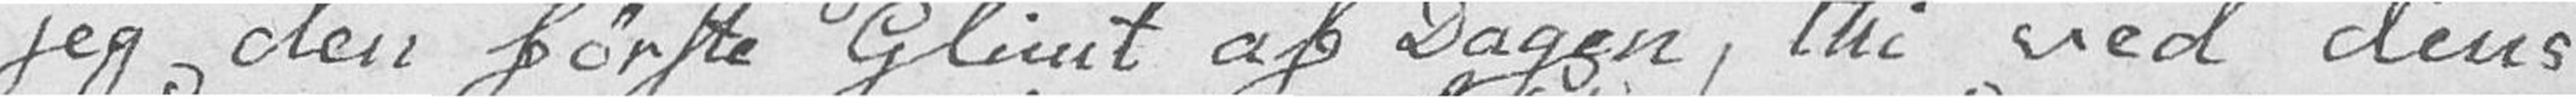jeg den første Glimt af Dagen, thi ved dens
